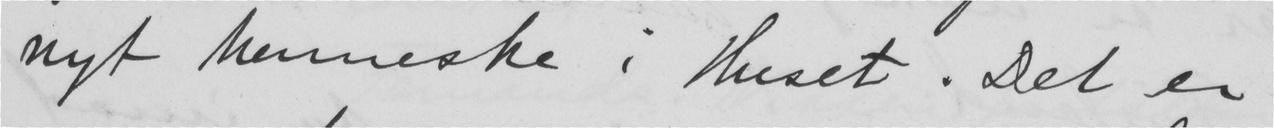nyt Menneske i Huset. Det er
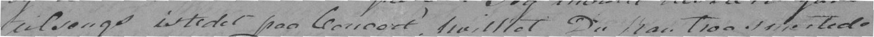tilsengs istedet paa Concert, hvilket Du kan troe smertede
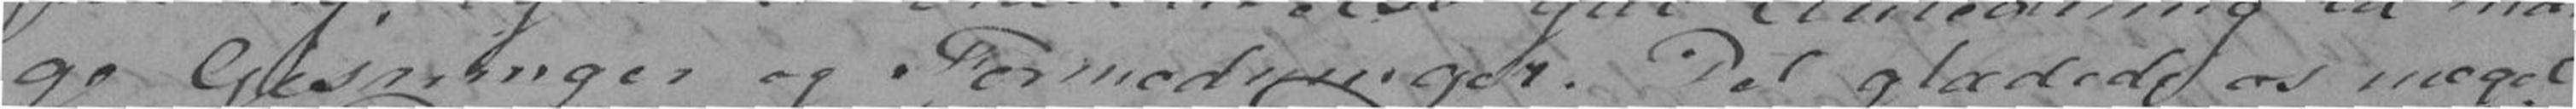ge Gisninger og Formodninger. Det glædede os meget
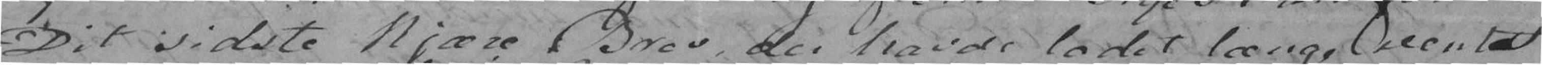Dit sidste kjære Brev, der havde ladet længe vente
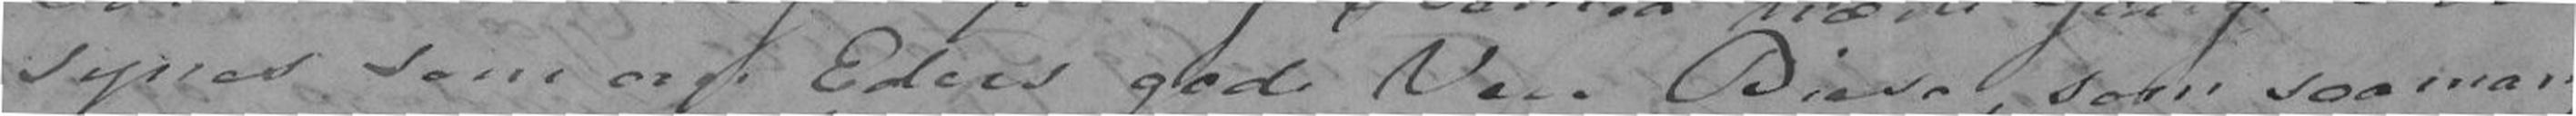synes som om Eders gode Ven Biese, som saa mange
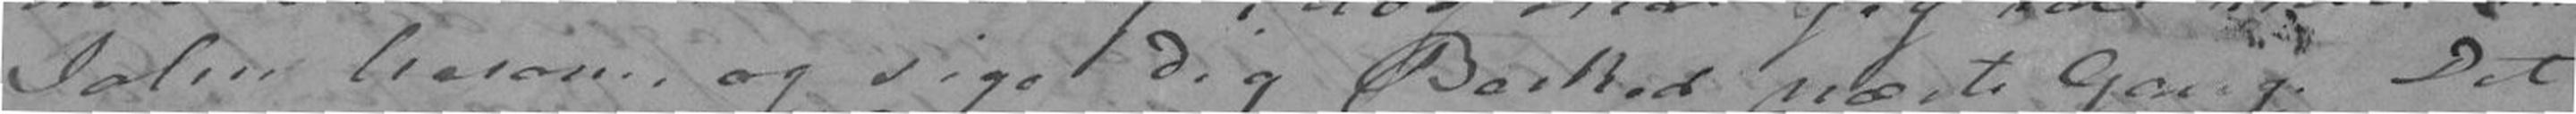John herom, og sige Dig Besked næste Gang. Det
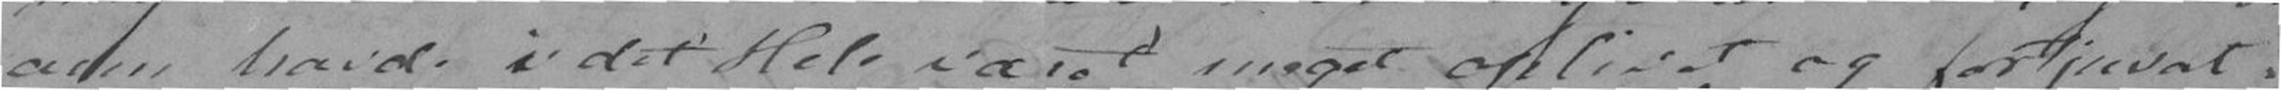cum havde i det Hele været meget oplivet og fortjuset.
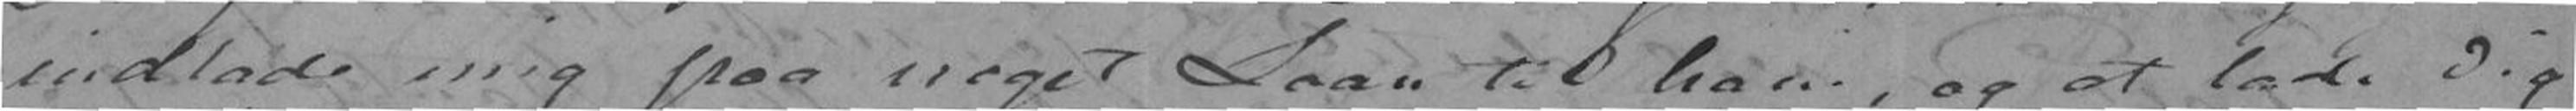indlade mig paa noget Laan til ham, og at lade Dig
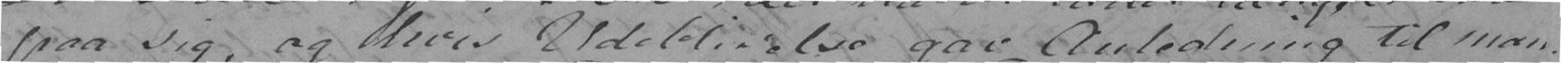paa sig, og hvis Udeblivelse gav Anledning til man-
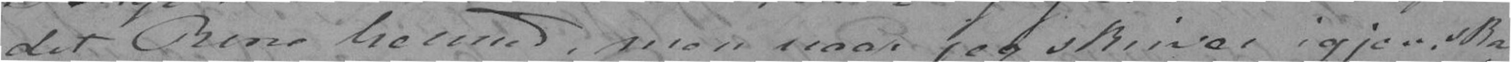det Rene hermed, men naar jeg skriver igjen, skal
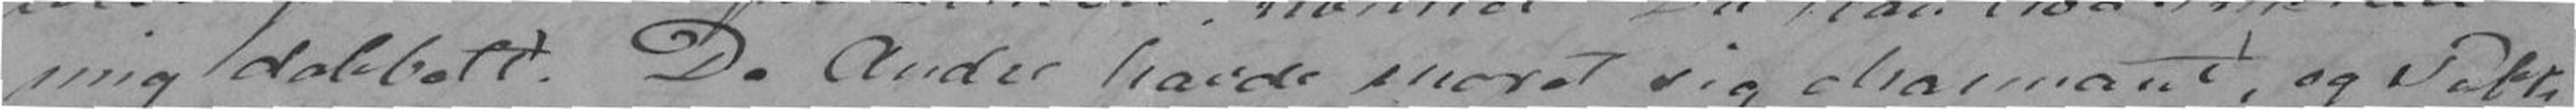mig dobbelt. De Ander havde moret sig charmant, og Publi-
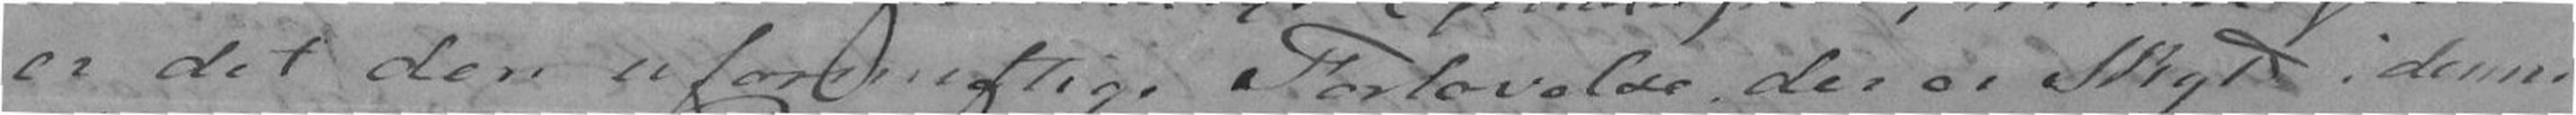er det den ufornuftige Forlovelse der er Skyld i denne
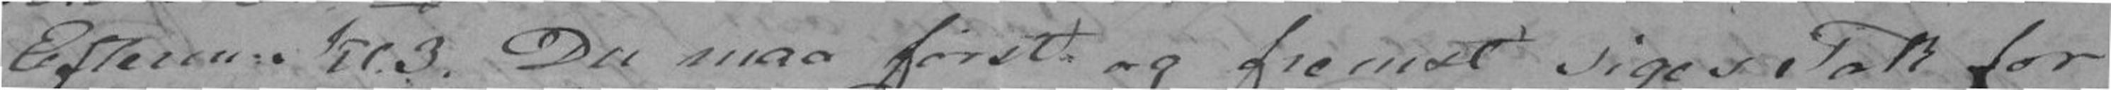Efterm. Kl.3. Du maa først og fremst siges Tak for
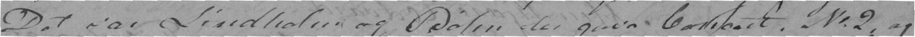Det var Lindholm og Bøhn der gave Concert. N.2, og
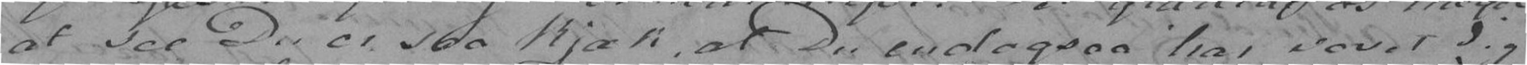at see Du er saa kjæk, at Du endogsaa gar vovet Dig
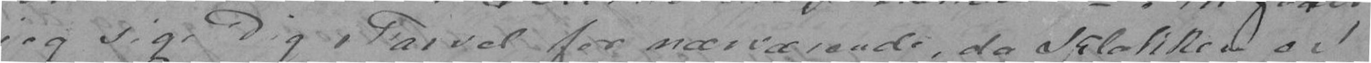jeg sige Dig Farvel for nærværende, da Klokken er
